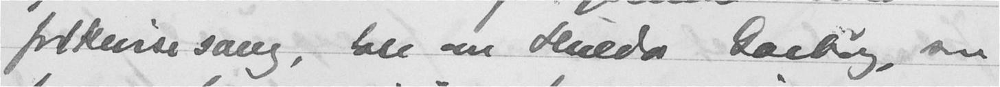folkevise sang, tale om Hulda Garborg, som
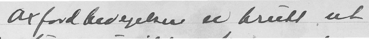Oxfordbevegelsen er brutt ut
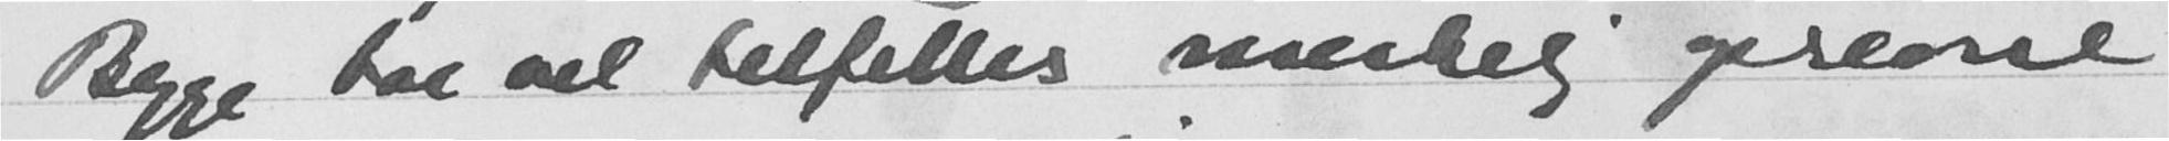Begge har vel tilfelles merkelig oprevne
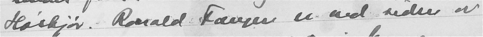Haskjør. Ronald Fangen er ved siden av
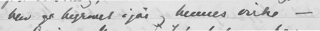blev  begravet igår og hennes virke -
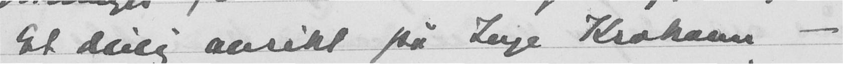Et deilig ansikt på Inge Krokann -
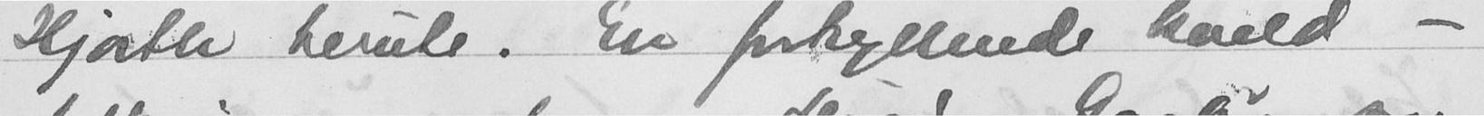Hjorth herute. En fortryllende kveld -
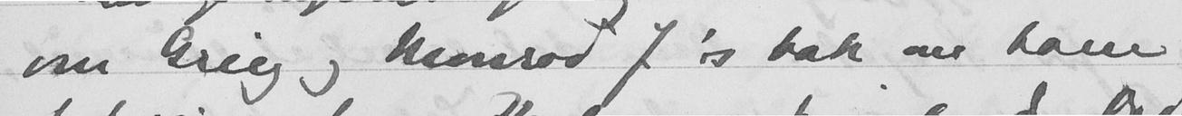om Grieg og Monrad J's bok om hans
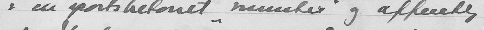i en sportsbetonet munter og offentlig
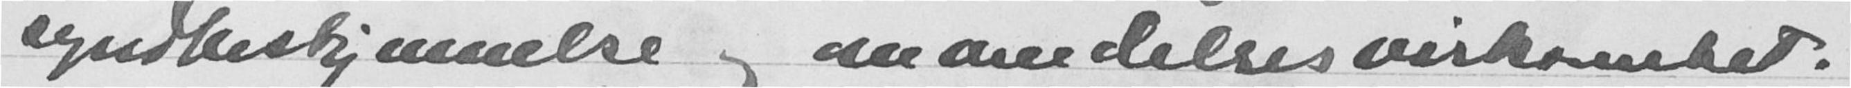syndbeskjennelse og omvendelsesvirksomhet.
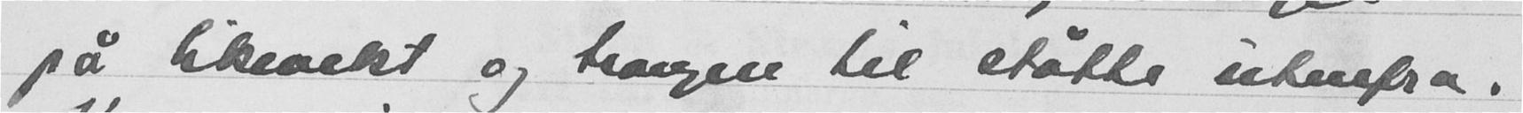på likevekt og trangen til støtte utenfra.
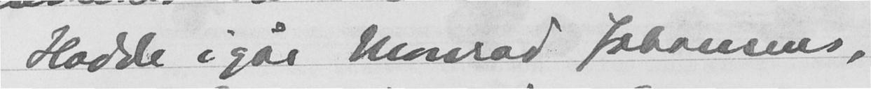Hadde igår Monrad Johansens,
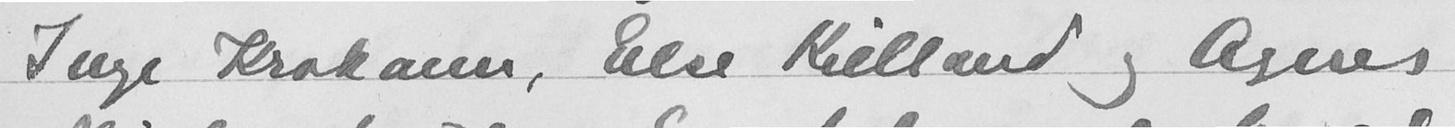Inge Krokann, Else Kielland og Agnes
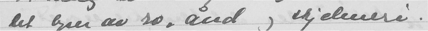det lyser av ro "ånd og skjelmeri.
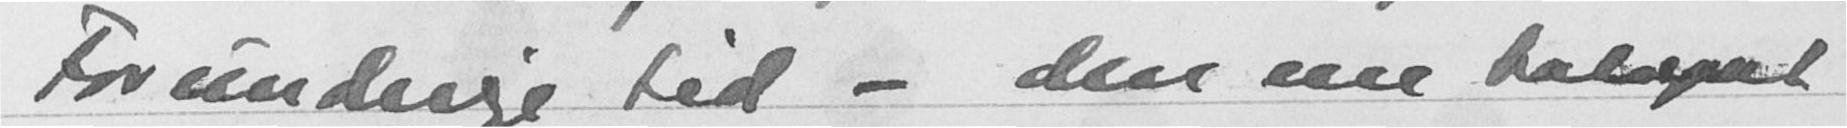Forunderlige tid - den ene balspnt
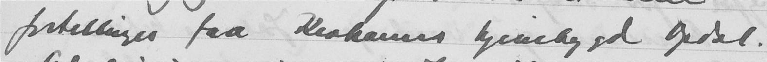fortillinger fra Krokanns hjembygd Opdal.
